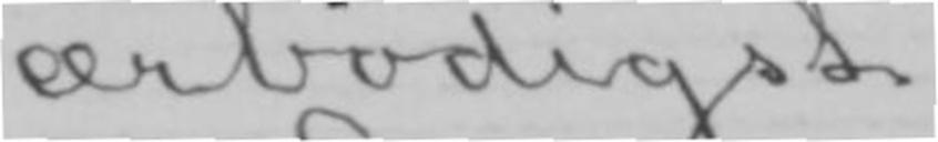ærbødigst
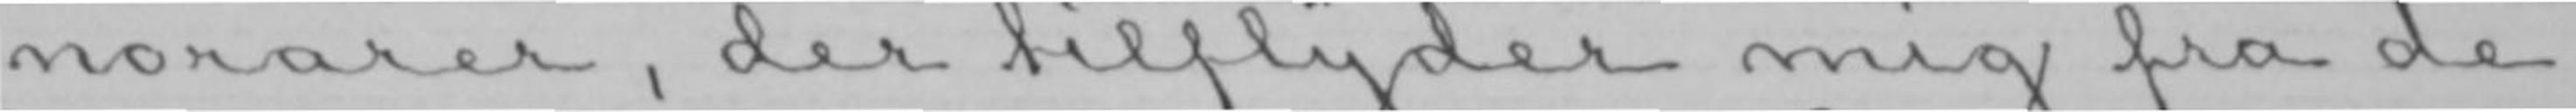norarer, der tilflyder mig fra de
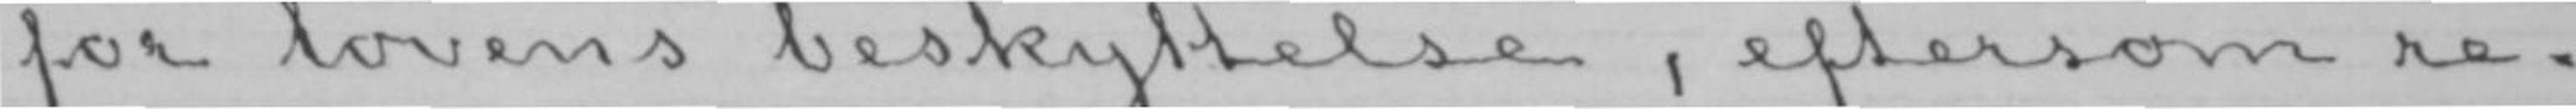for lovens beskyttelse, eftersom re-
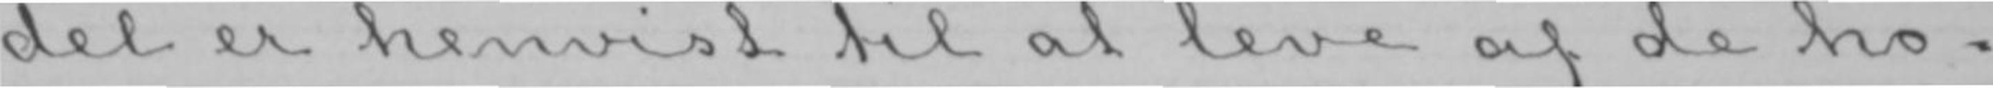del er henvist til at leve af de ho-
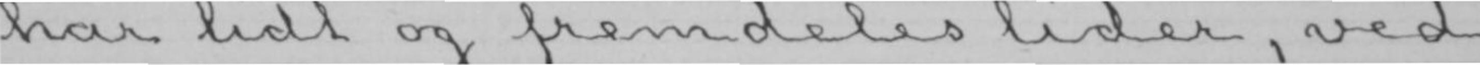har lidt og fremdeles lider, ved
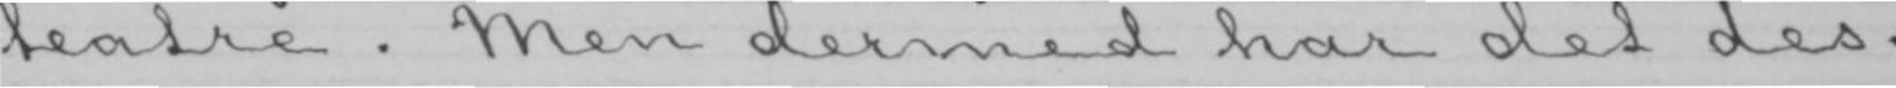teatre. Men dermed har det des-
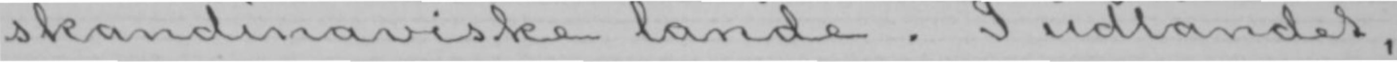skandinaviske lande. I udlandet,
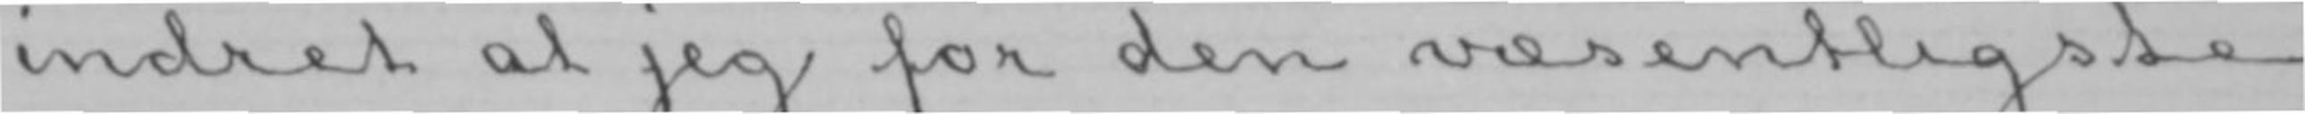indret at jeg for den væsentligste
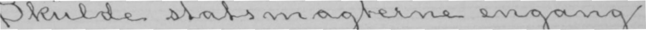Skulde statsmagterne engang
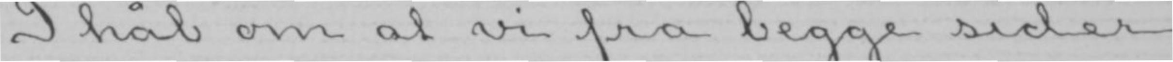I håb om at vi fra begge sider
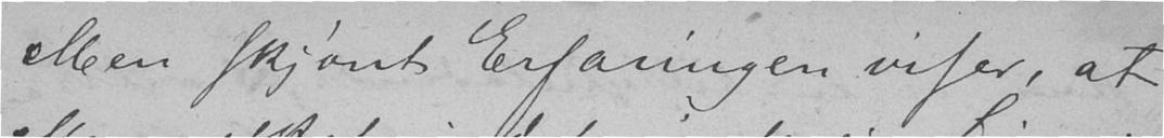Men skjønt Erfaringen viser, at
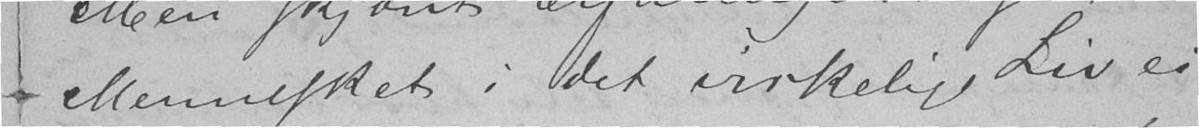Mennesket i det virkelige Liv ei
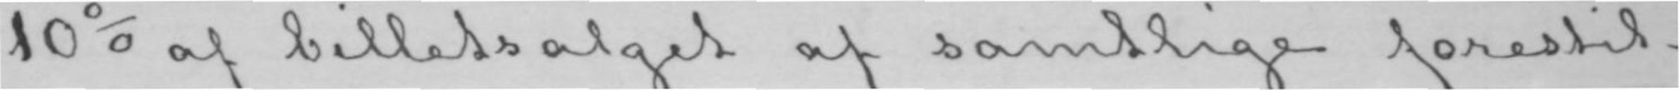10 % af billetsalget af samtlige forestil-
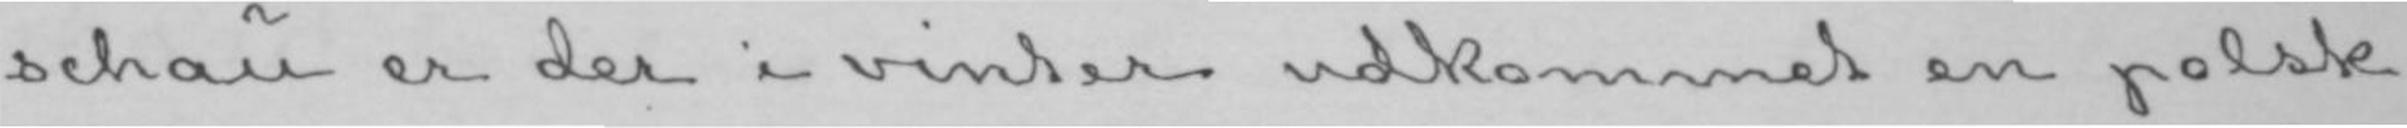schau er der i vinter udkommet en polsk
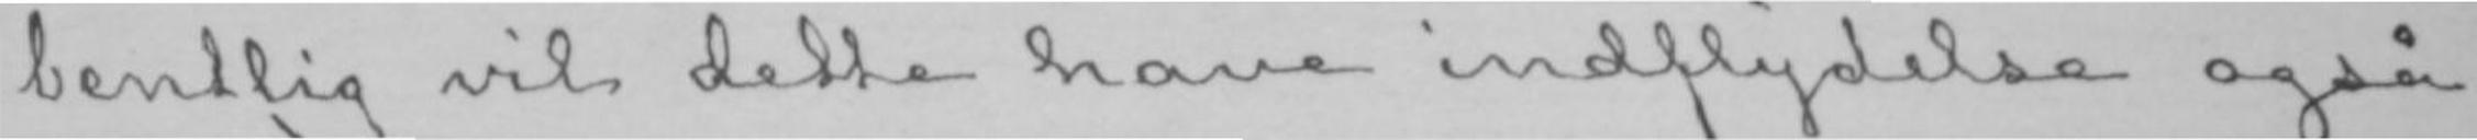bentlig vil dette have indflydelse også
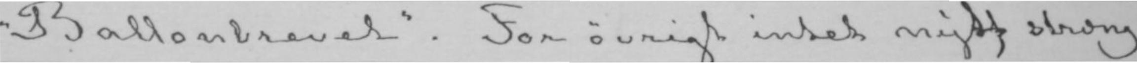«Ballonbrevet». For øvrigt intet nytt; stræng
